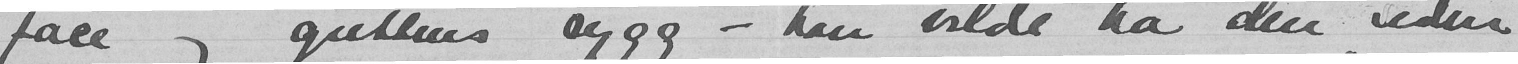face og guttens rygg - han vilde ha den siden
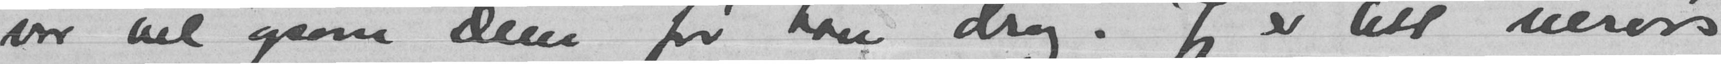var vel opom Dem før han drog. Jeg er litt nervøs
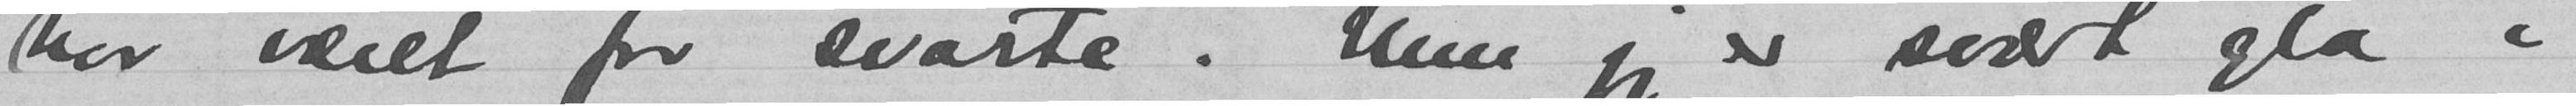har været for svarte. Men jeg er svært gøa o
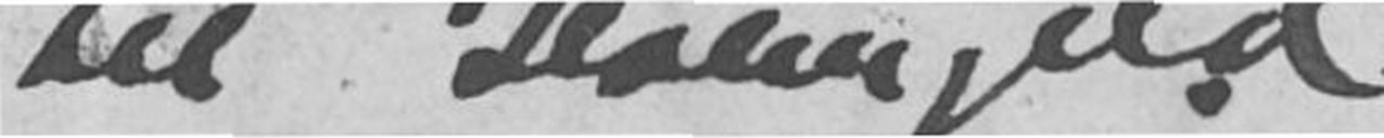til Homgjeld
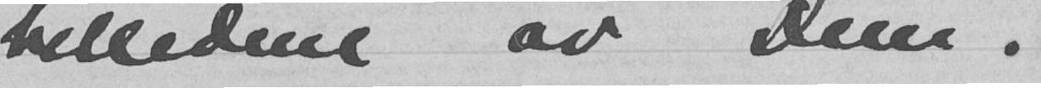billedene av Dem.
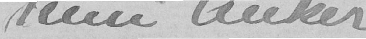Nini Anker
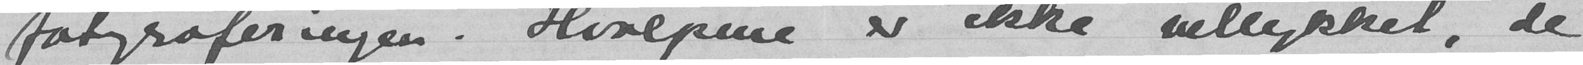fotograferingen. Hvalpene er ikke vellykket, de
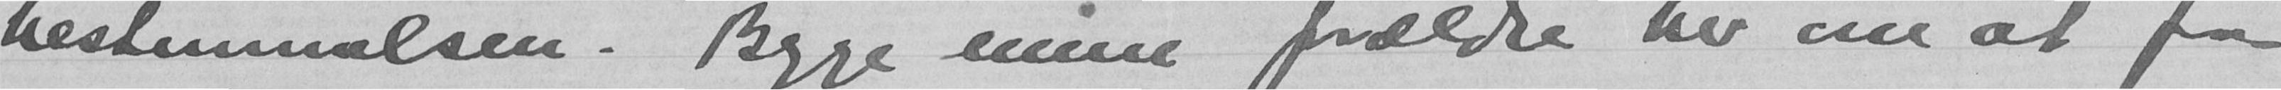bestemmelsen. Begge mine forældre ber om at faa
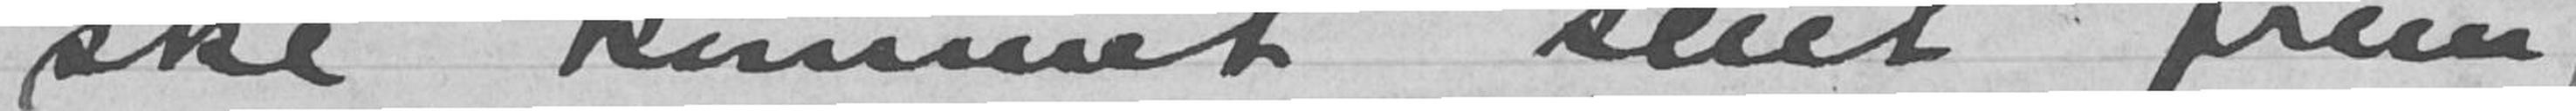ske kommet sent frem
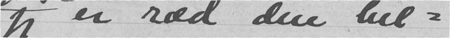jeg er ræd den bil-
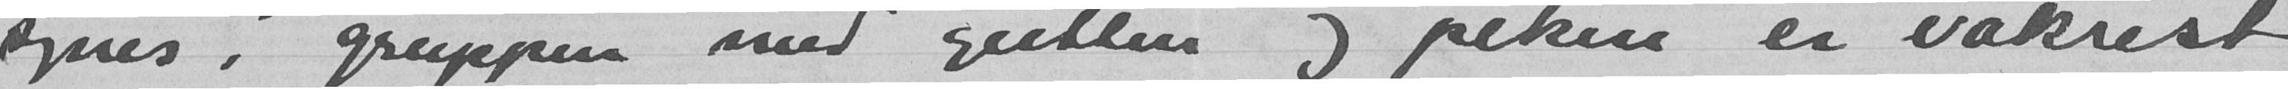synes, gruppen med gutten og piken er vakrest
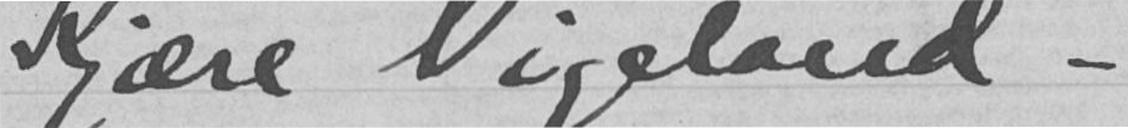Kjære Vigeland -
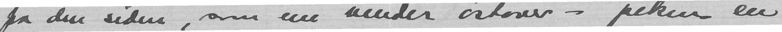fra den siden, som nu vender østiver - piken en
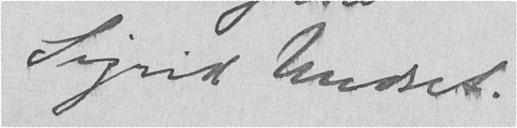Sigrid Undset.
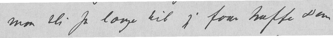maa bli for længe til jeg faar træffe Dem
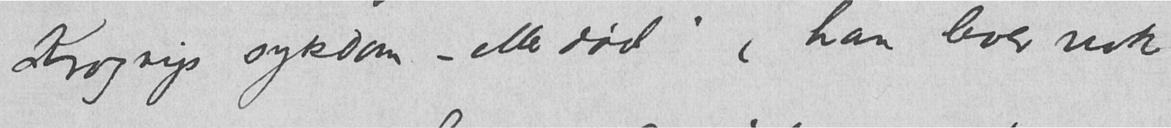Krogvigs sykdom – eller død" (han lever nok
